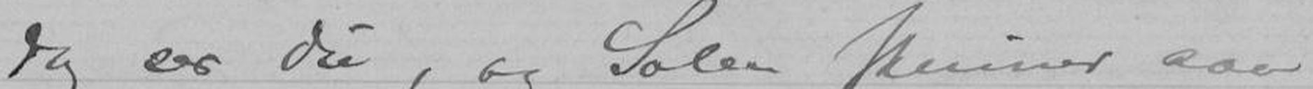dag ser du, og Solen skinner saa
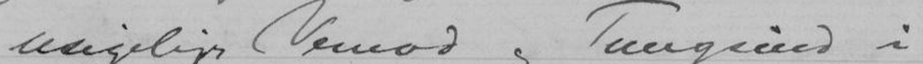usigelige Vemod og Tungsind i
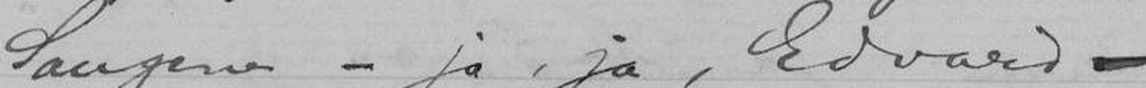Sangene - ja, ja, Edvard ---
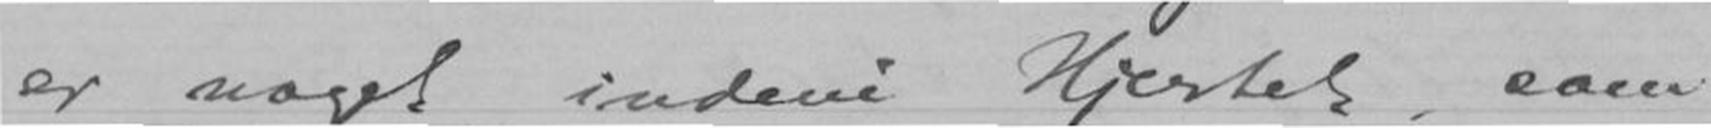er noget indeni Hjertet, som
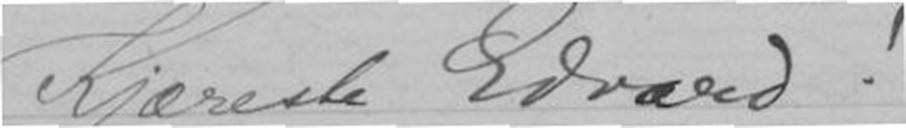Kjæreste Edvard!
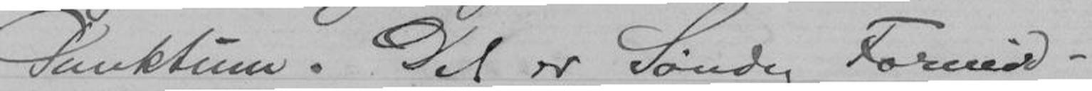Punktum. Det er Søndag Formid-
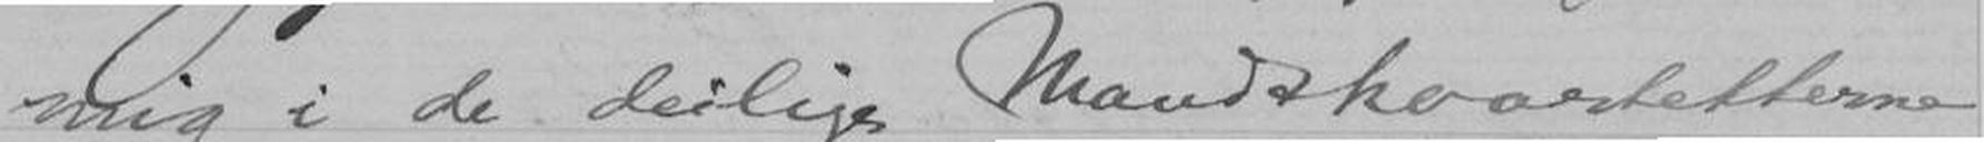mig i de deilige Mandskvartetterne
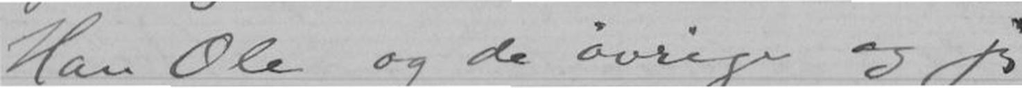Han Ole og de øvrige og jeg
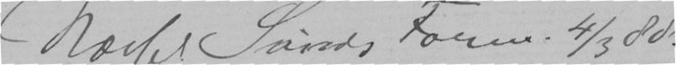Næsset Søndag Form. 4/3 88.
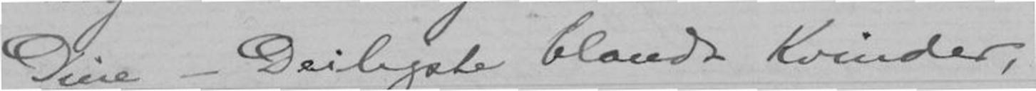Dine - Deiligste blandt Kvinder,
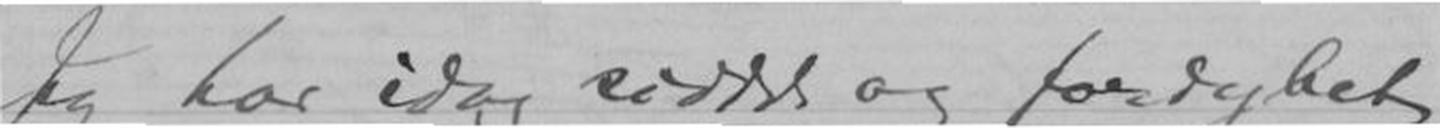Jeg har idag siddet og fordybet
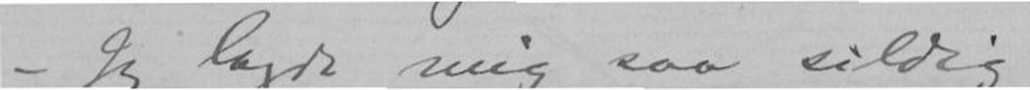- Jeg lagde mig saa sildig -
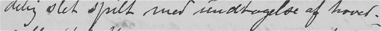delig slet spilt med undtagelse af hoved-
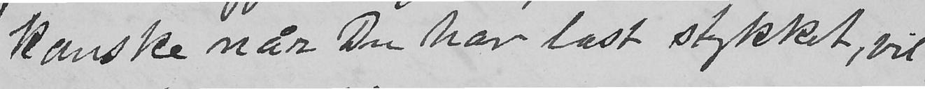kanske når Du har læst stykket, vil
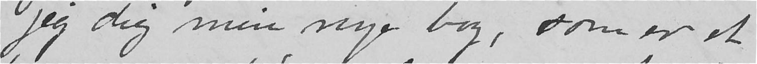jeg dig min nye bog, som er et
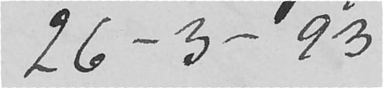26-3-93
